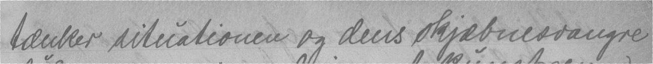tænker situationen og dens skjæbnesvangre
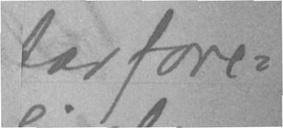tar fore-
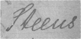Steens
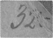32
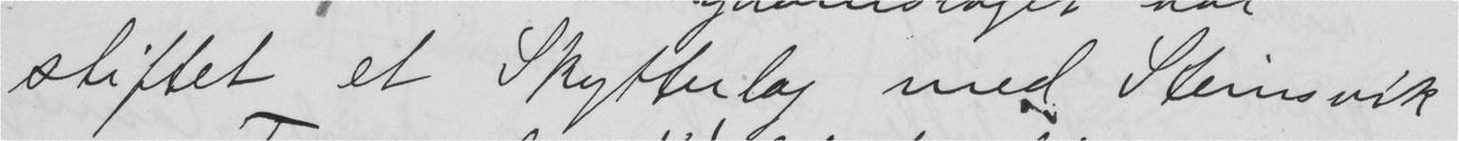stiftet et Skytterlag med Steinsvik
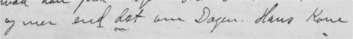og mer end det om Dagen. Hans Kone
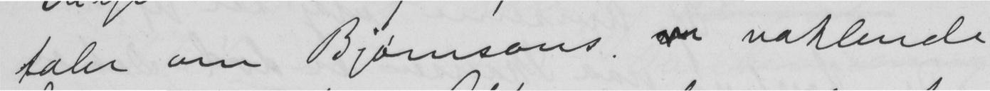taler om Bjørnsons  vaklende
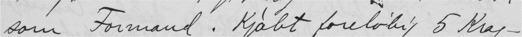som Formand. Kjøbt foreløbig 5 Krag-
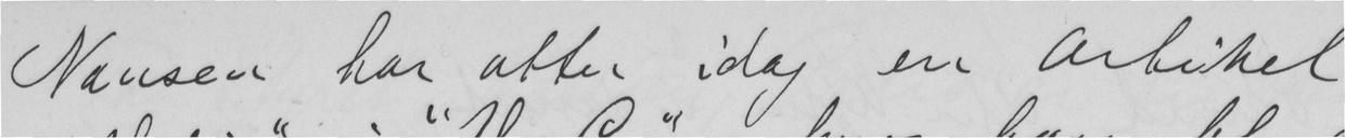Nansen har atter idag en Artikel
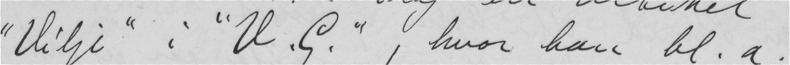"Vilje" i "V G.", hvor han bl.a.
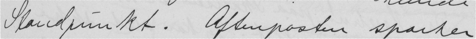Standprunkt. Aftenposten sparker
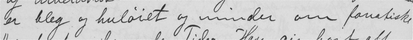er bleg og huløiet og minder om fanatiske
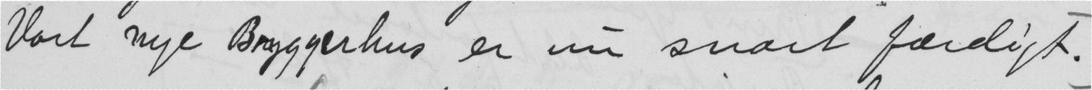Vort nye Bryggerhus er nu snart færdigt.
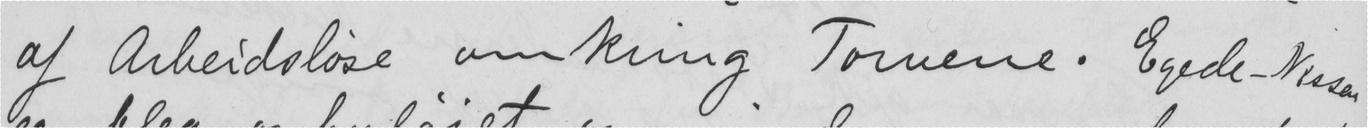af Arbeidsløse omkring Torvene. Egede-Nissen
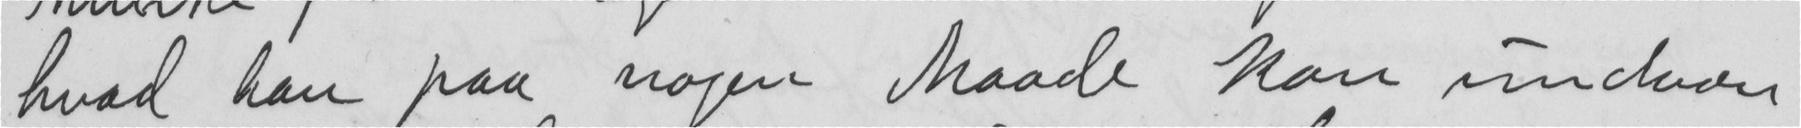hvad han paa nogen Maade kan undvære
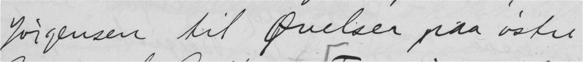Jørgensen til Øvelser paa østre
